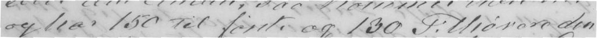og har 150 til første og 130 Tilhørere den
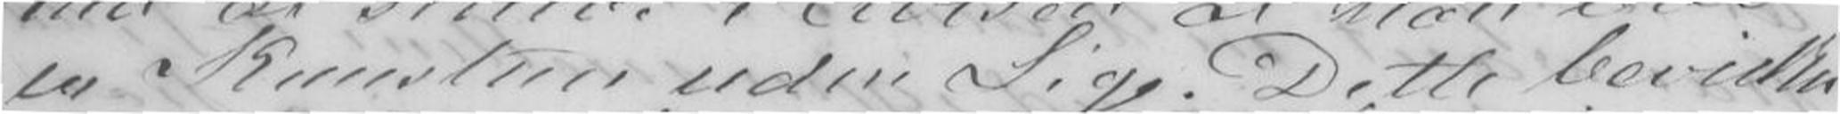en Kunstner uden Lige. Dette bevirker
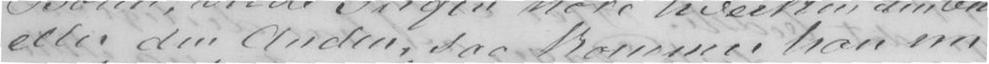eller den Anden, saa kommer han nu
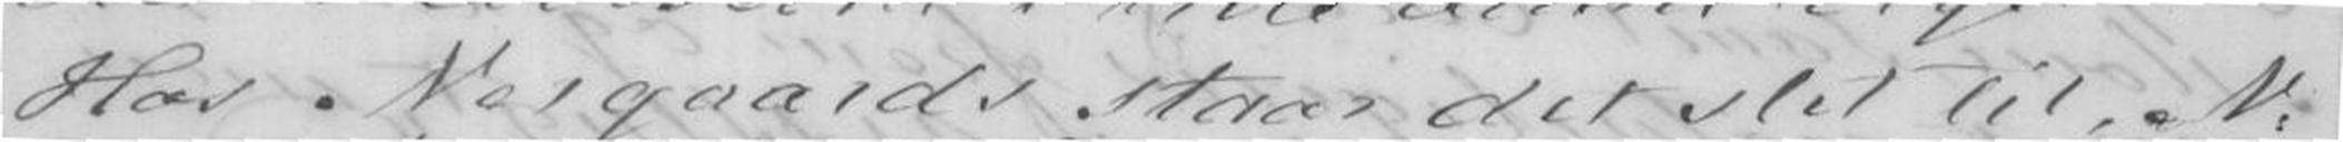Hos Nergaards staar det slet til, N:
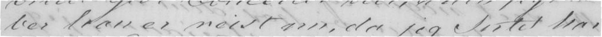ber han er reist nu, da jeg Intet har
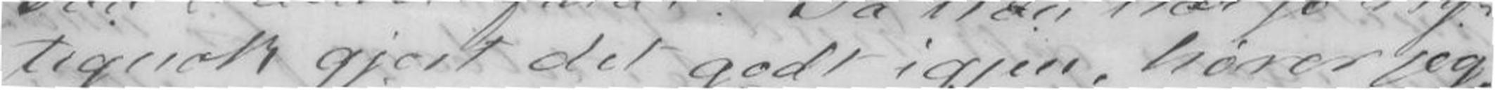tignok gjort det godt igjen, hører jeg,
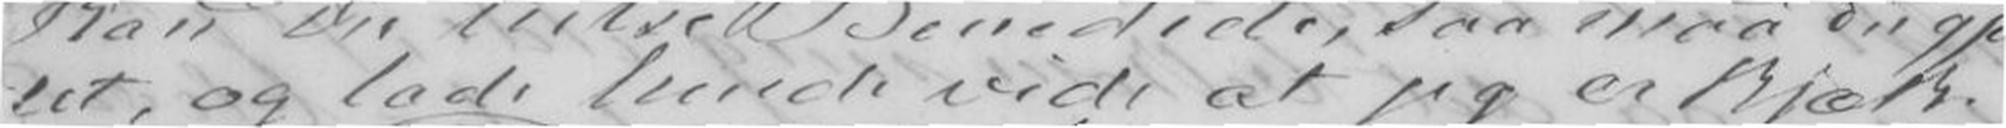ret, og lade hende vide at jeg er kjæk.
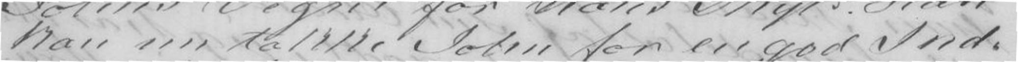kan nu takke John for en god Ind-
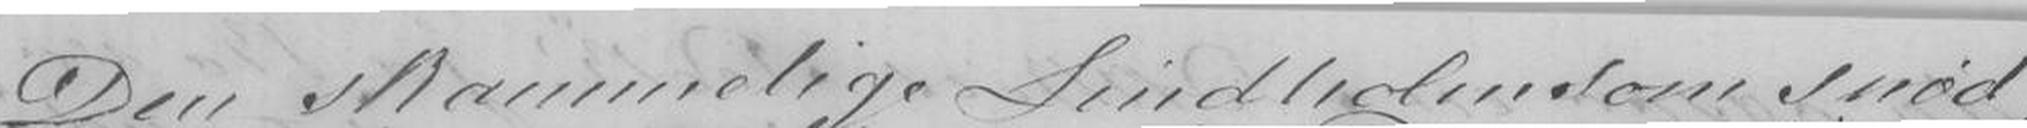Den skammelige Lindholm som snød
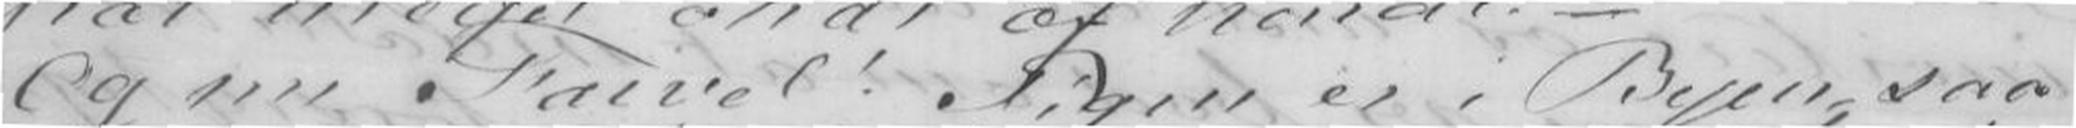Og nu Farvel! Pigen er i Byen sa
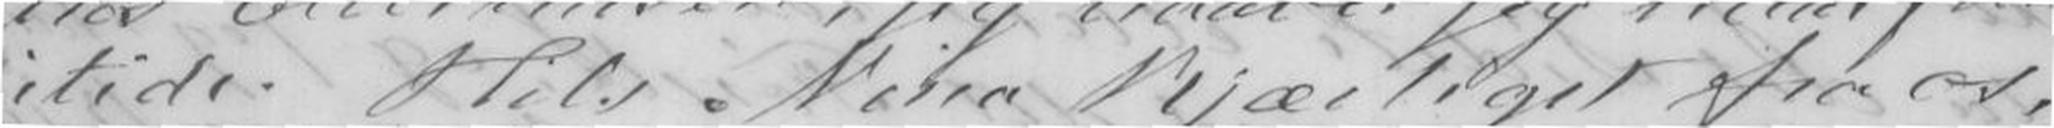itide. Hils Nina kjærligt fra os,
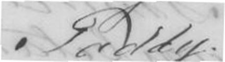Paddy.
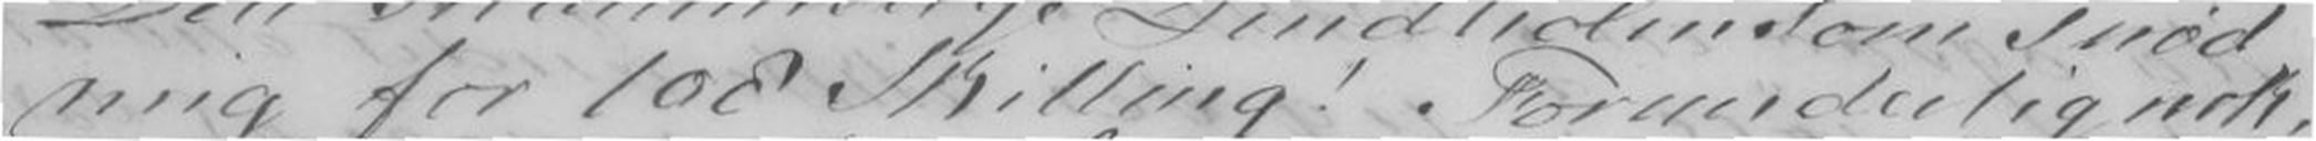mig for 108 Skilling! Forunderlig nok,
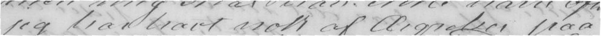jeg har havt nok af Ærgrelser paa
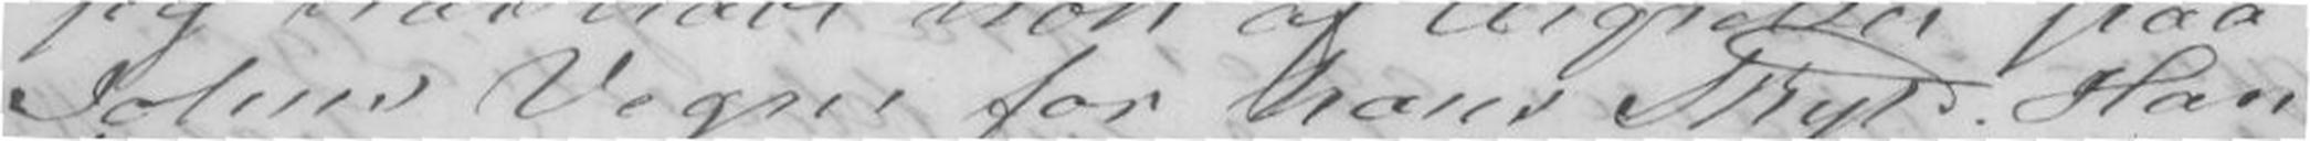Johns Vegne for hans Skyld. Han
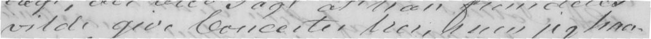vilde give Concerter her, men jeg haa-
